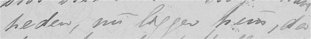heden, nu ligger hun, da
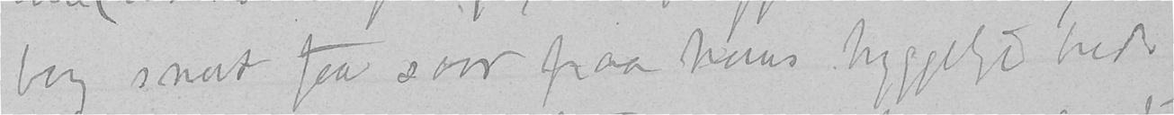borg snart faa svar paa hans hyggelige brev.
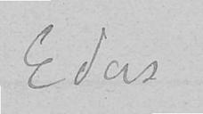Eders
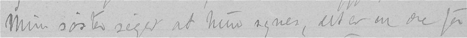Min søster siger at hun synes, det er en ære for
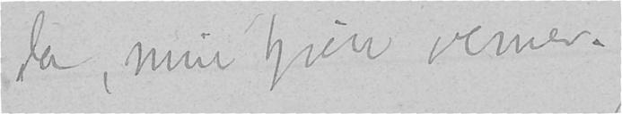da, mine kjære venner.
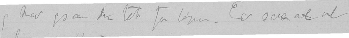og tror ogsaa det tak for bogen. Lev saa al vel
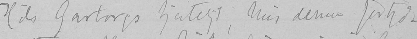Hils Garborgs hjerteligt, hvis denne forkjø-
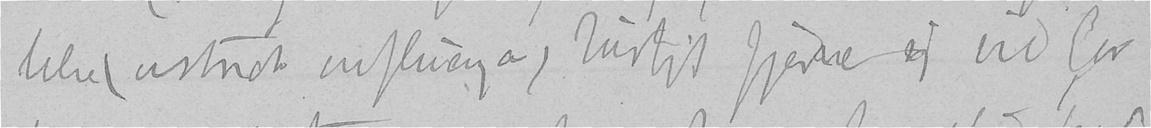lelse (vistnok influensa) hurtigt fjerne sig vil Gar-
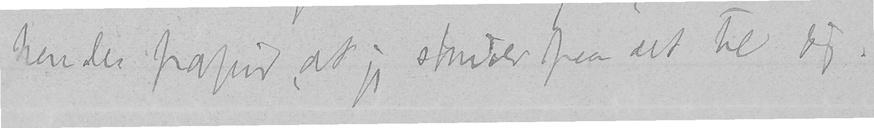hendes papir, at jeg skriver paa det til dig.
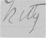Kitty
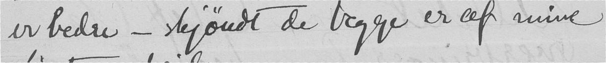er bedre - skjøndt de begge er af mine
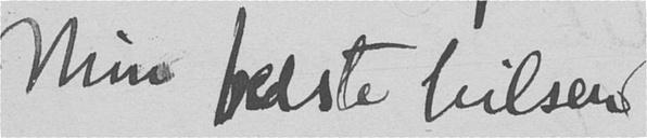Min bedste hilsen
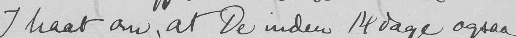I haab om, at De inden 14 dage ogsaa
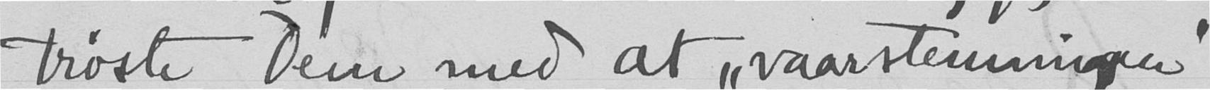trøste Dem med at "vaarstemningen"
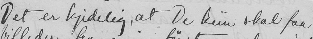.Det er kjedelig, at De kun skal faa
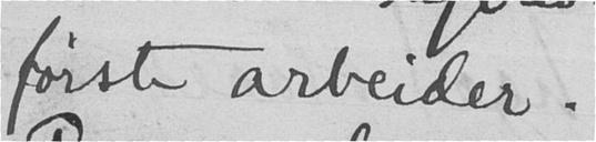første arbeider.
